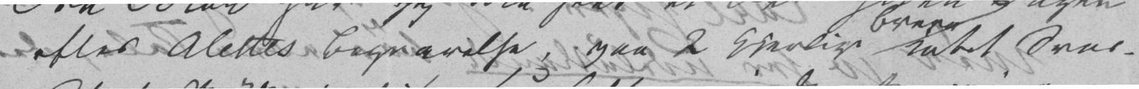efter Alettes Begravelse, paa 2 kjerlige Breve intet Svar.
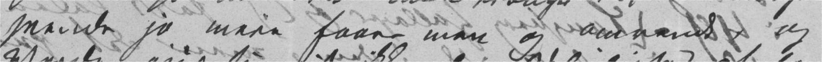seende jo mere faaer man og omvendt, og
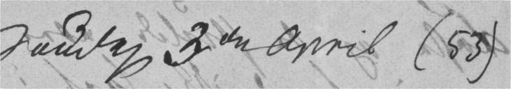Søndag 3die April
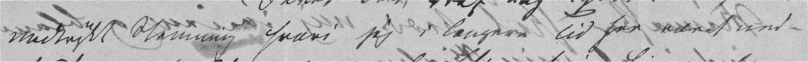nedtrykt Stemning hvori jeg i længere Tid har været ned-
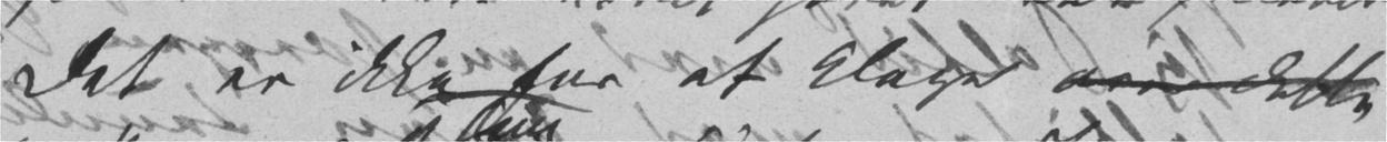Det er ikke for at klage over dette
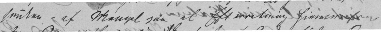sunken – af Mangel paa al Efterretning hjemmefra.
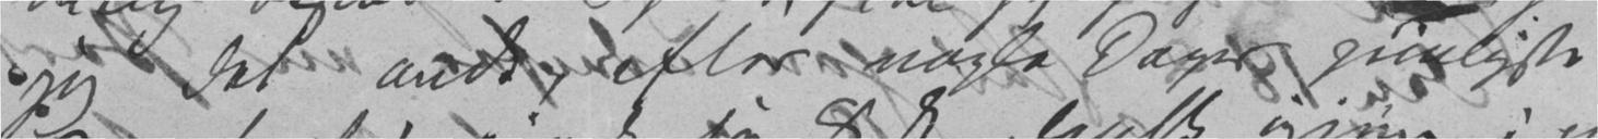jeg det ondt, efter nogle Dages piinligste
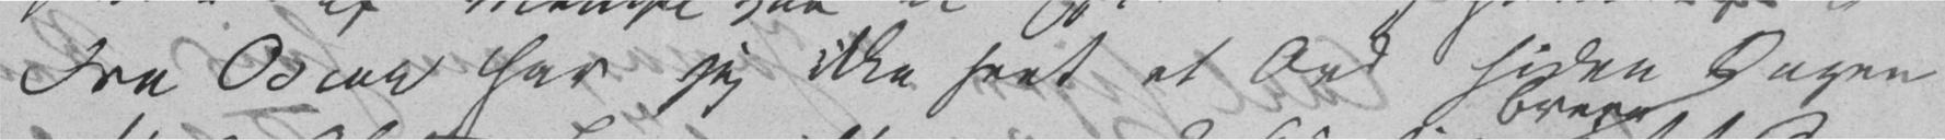Fra Oscar har jeg ikke seet et Ord siden Dagen
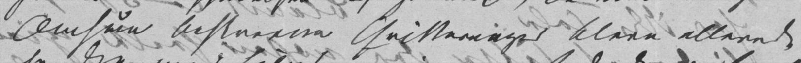Omhue beskrevne Qvitteringer bleve allerede
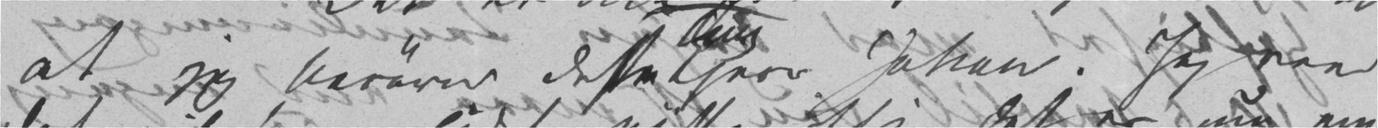at jeg berører disse Ting Kjere Johan. Jeg veed
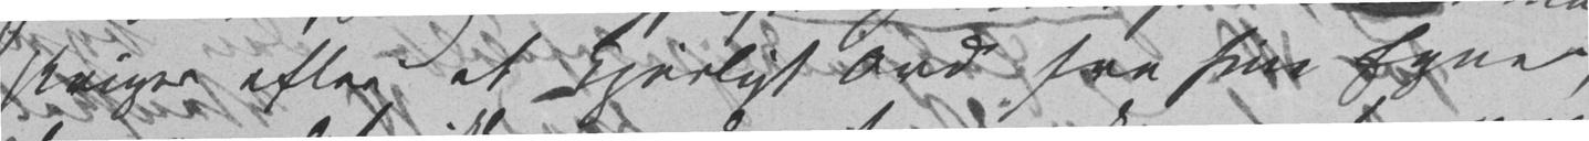skriger efter et kjerligt Ord fra sine Egne,
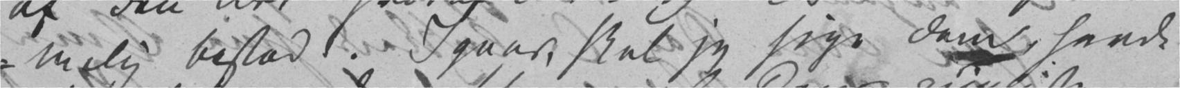-melig bestod. Igaar, skal jeg sige Dem, havde
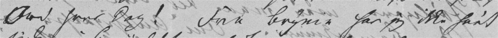Ord hver Dag! Fra Brynie har jeg ikke hørt
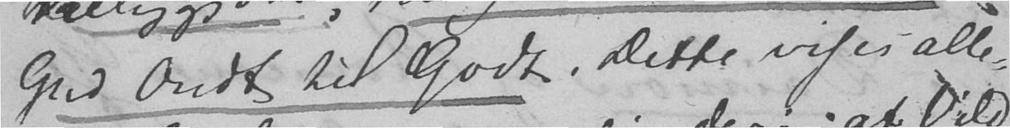Gud Ondt til Godt. Dette vises alle-
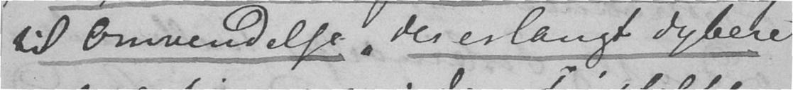til Omvendelse, der er langt dybere
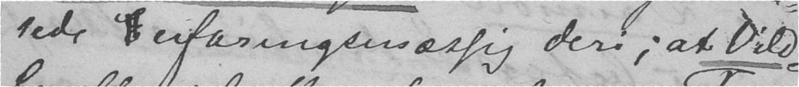rede erfaringsmæssig deri, at Vild
